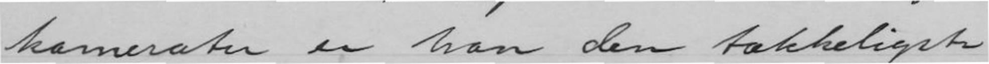kamerater er han den tækkeligste
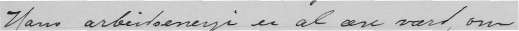Hans arbeidsenergi er al ære vært, om
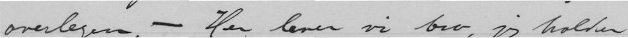overlegen. - Her lever vi bra, jeg holder
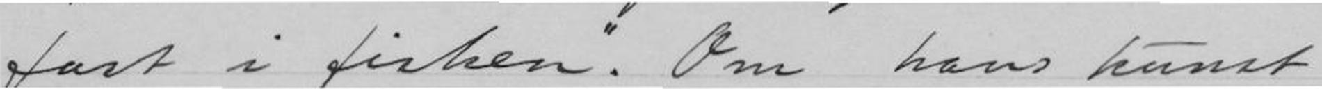"fast i fisken". Om hans kunst
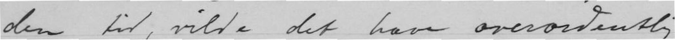den tid, vilde det have overordentlig
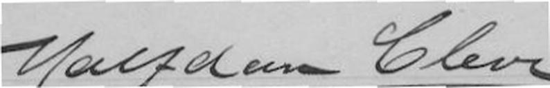Halfdan Cleve
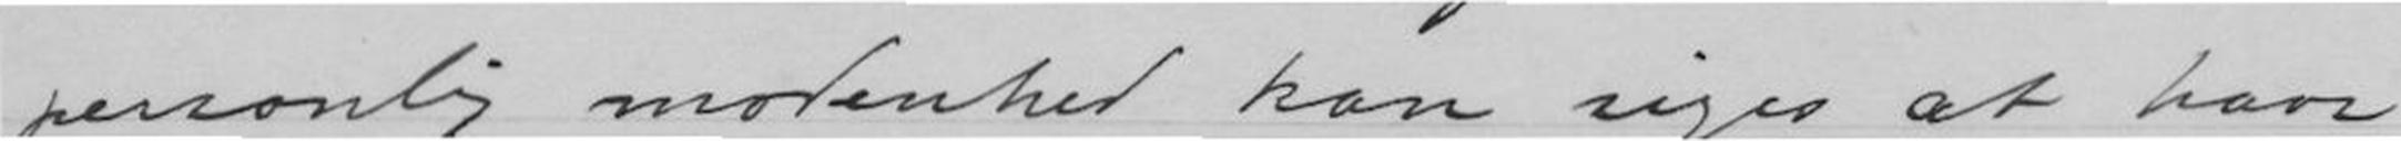personlig modenhed kan siges at have
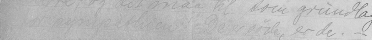for sympathien! De er søde, er de. -
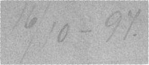16/10-97.
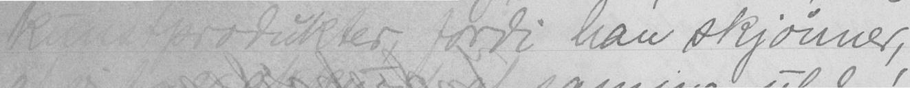kunstprodukter, fordi han skjønner,
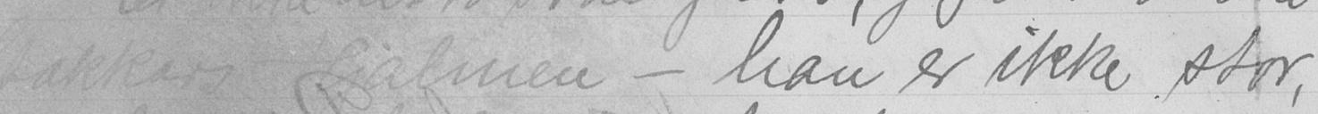Stakkars Hjalmen - han er ikke stor,
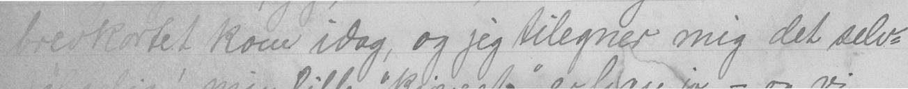brevkortet kom idag, og jeg tilegner mig det selv-
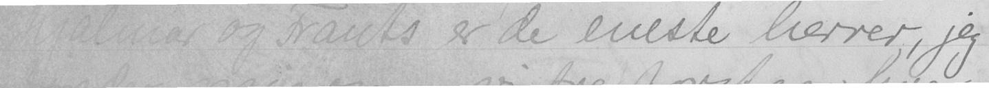Hjalmar og Frants er de eneste herrer, jeg
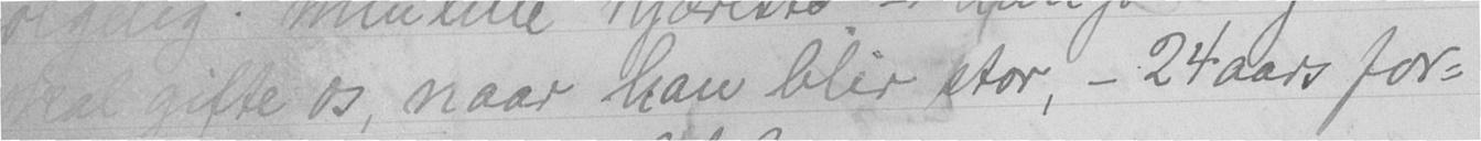skal gifte os, naar han blir stor, - 24 aars for-
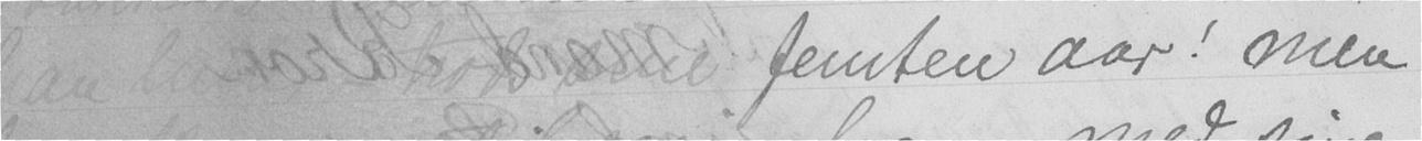han  trods sine femten aar! men
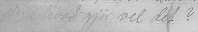skjel, hvad gjør vel det?
